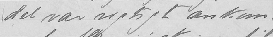det var rigtigt ankom-
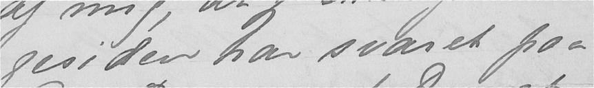gesiden har svaret paa
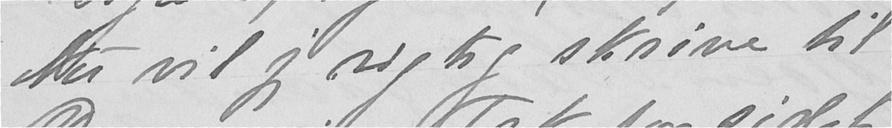Nu vil jeg rigtig skrive til
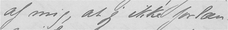af mig, at jeg ikke for læn-
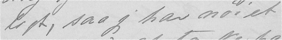ligt, saa jeg har nøiet
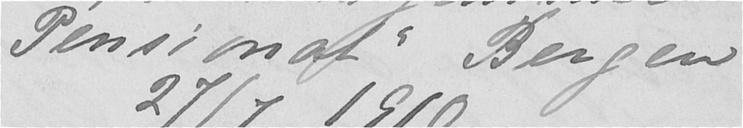Pensionat" Bergen
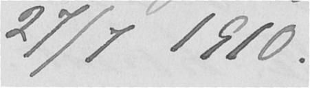27/7 1910.
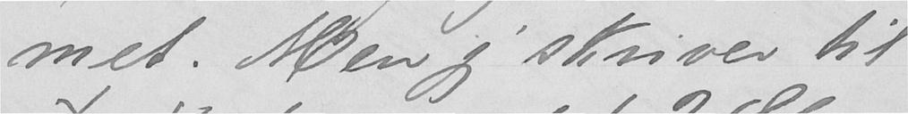met. Men jeg skriver til
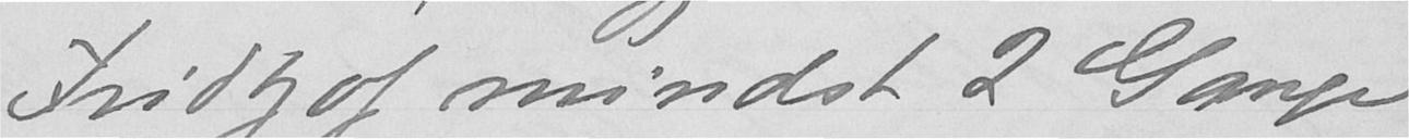Fridtjof mindst 2 Gange
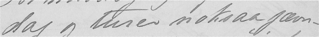dag og turer noksaa jævn-
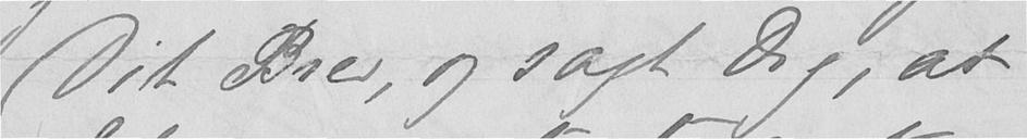Dit Brev, og sagt Dig, at
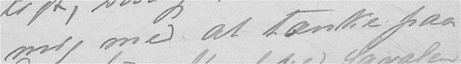mig med at tænke paa
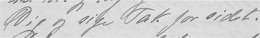Dig og sige Tak for sidst.
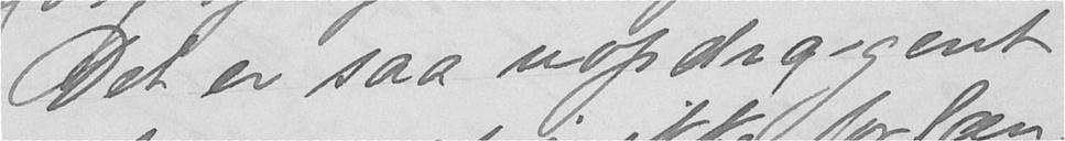Det er saa uopdragent
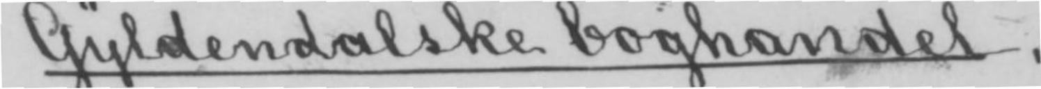Gyldendalske boghandel,
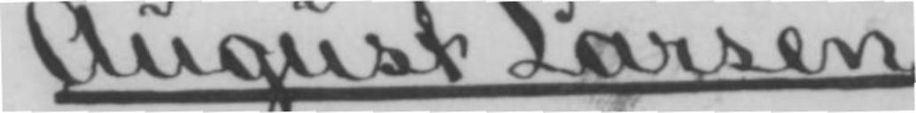August Larsen,
